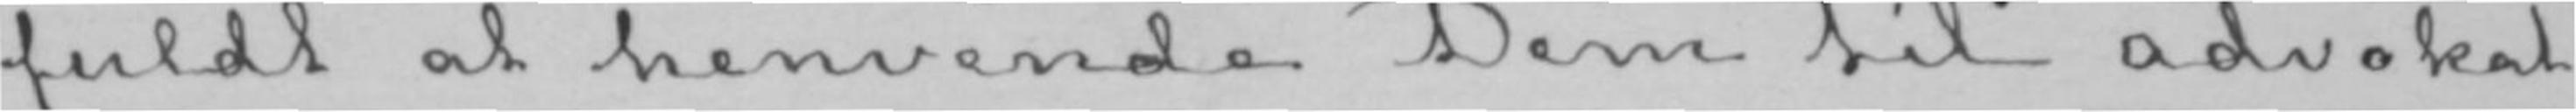fuldt at henvende Dem til advokat
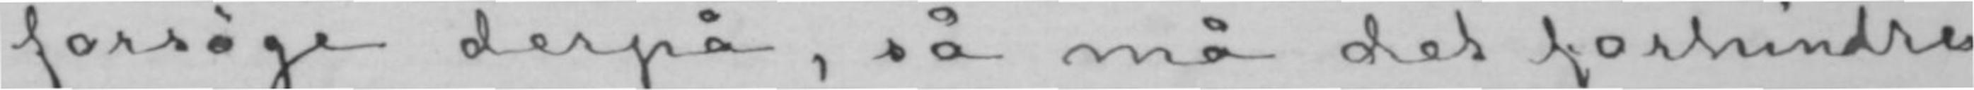forsøge derpå, så må det forhindres
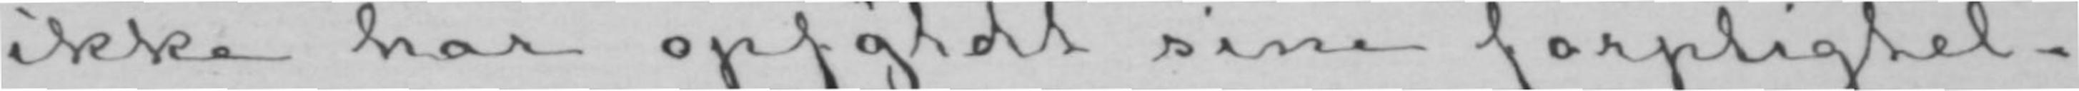ikke har opfyldt sine forpligtel-
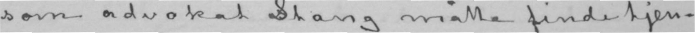som advokat sStang måtte finde tjen-
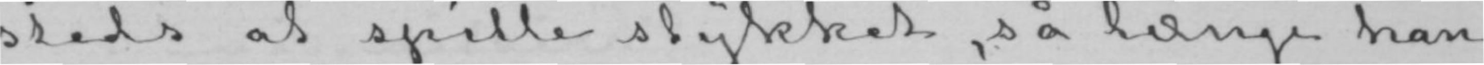steds at spille stykket, så længe han
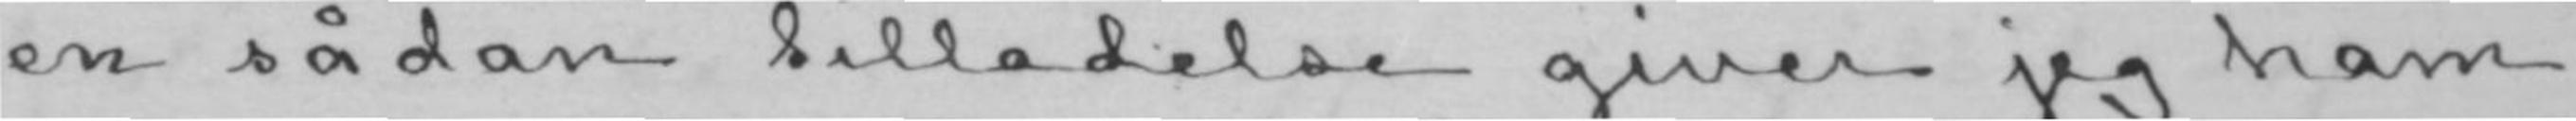en sådan tilladelse giver jeg ham
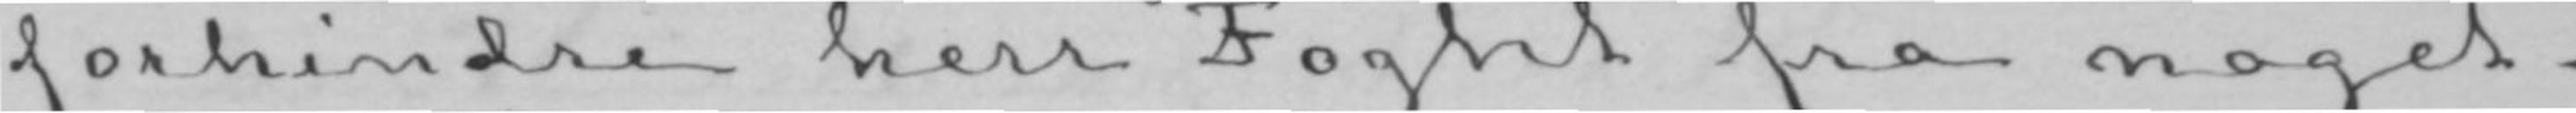forhindre herr Foght fra noget-
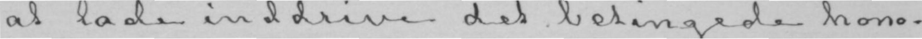at lade inddrive det betingede hono-
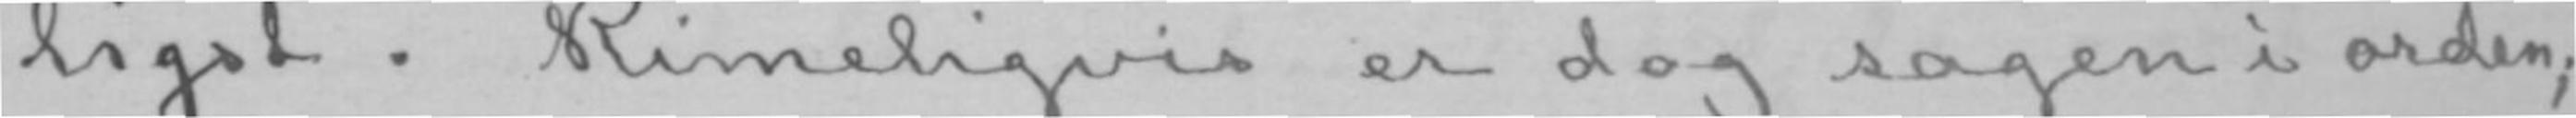ligst. Rimeligvis er dog sagen i orden;
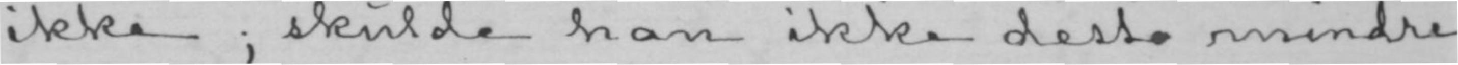ikke; skulde han ikke desto mindre
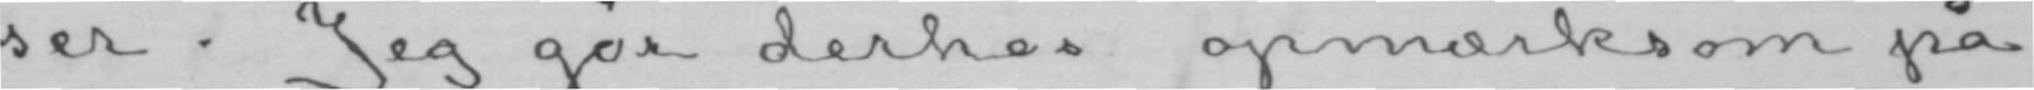ser. Jeg gør derhos opmærksom på
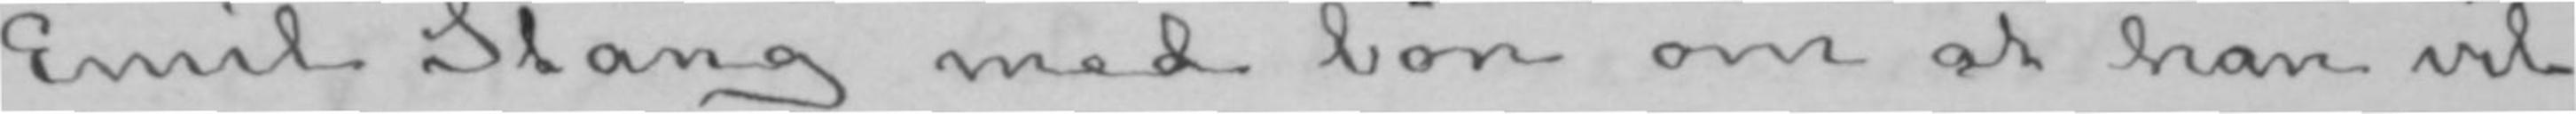Emil Stang med bøn om at han vil
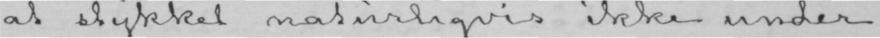at stykket naturligvis ikke under
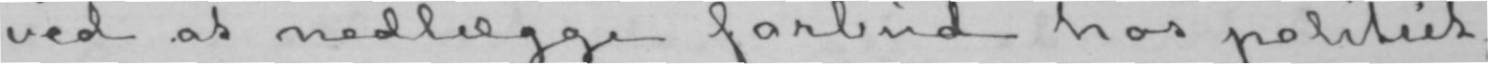ved at nedlægge forbud hos politiet,
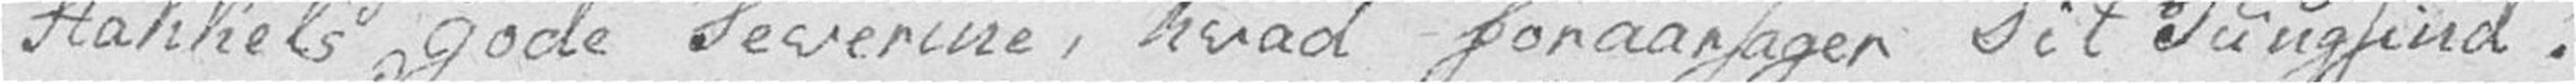Stakkels gode Severine, hvad foraarsager Dit Tungsind?
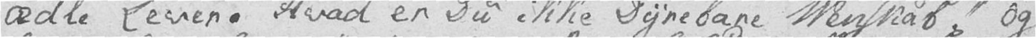ædle Lever. Hvad er Du ikke Dyrebare Venskab! Og
